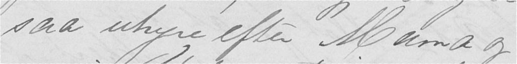saa uhyre efter Mama og
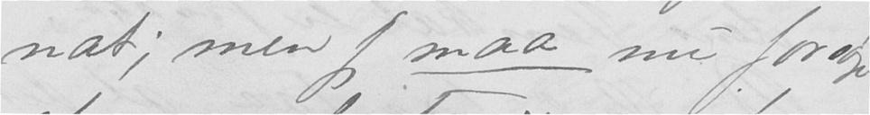nat; men jeg maa nu forsøge
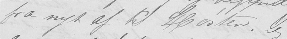fra nyt af til Høsten. Jeg
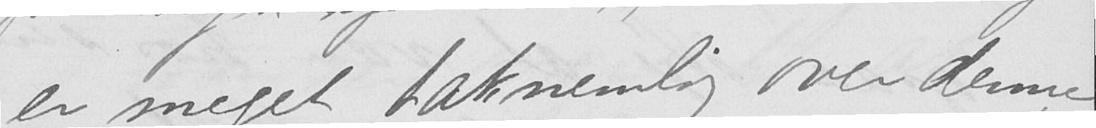er meget taknemlig over denne
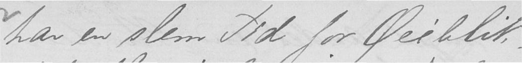har en slem Tid for Øieblik-
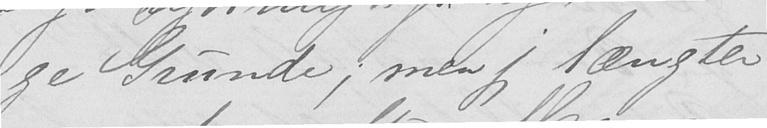ge Grunde; men jeg længter
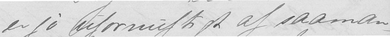er jo ufornuftigt af saaman-
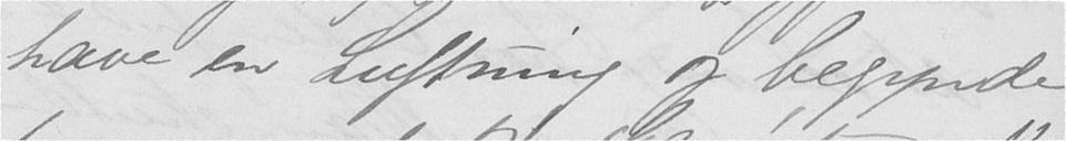have en Luftning og begynde
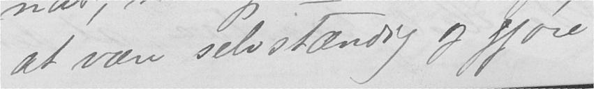at være selvstændig og gjøre
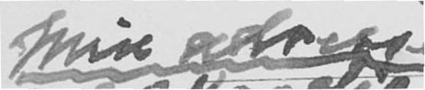Min adresse:
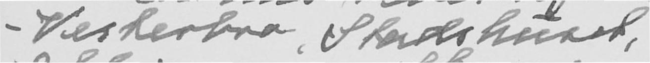- Vesterbro, Stadshuset
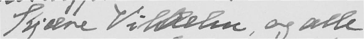Kjære Vilhelm og alle
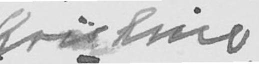Kristine
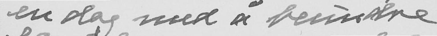en dag med å beundre
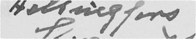Helsingfors
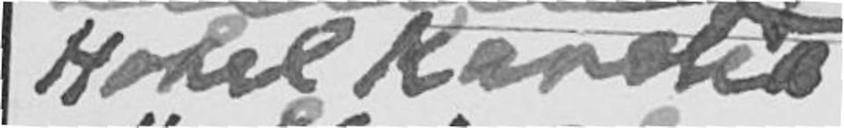Hotel Kares
